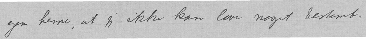egen herre, at jeg ikke kan lave noget bestemt.
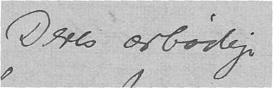Deres ærbødige
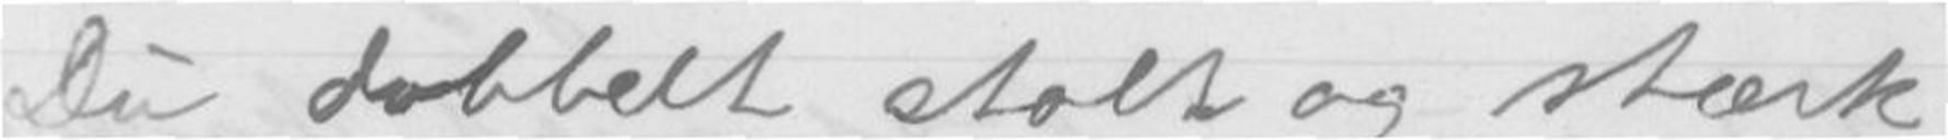Du dobbelt stolt og stærk
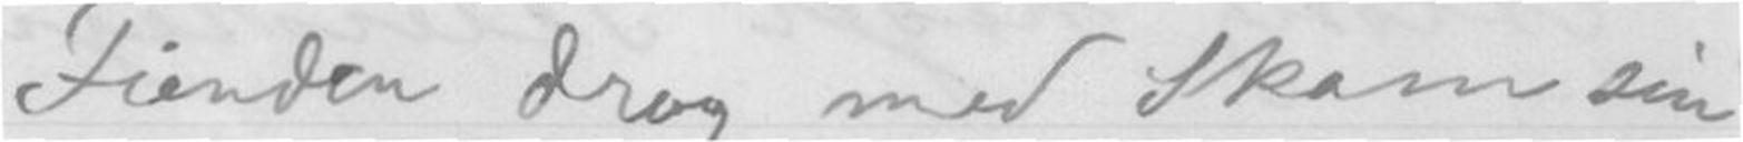Fienden drog med Skam sin
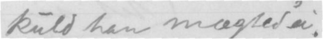kuld han magted ei.
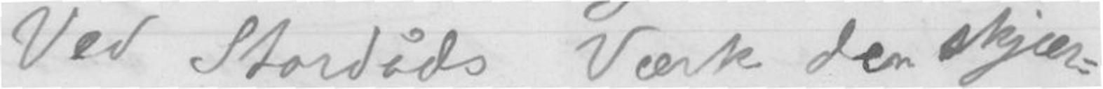Ved Stordåds Værk den skjær-
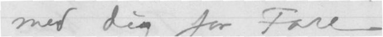med dig for Fare.
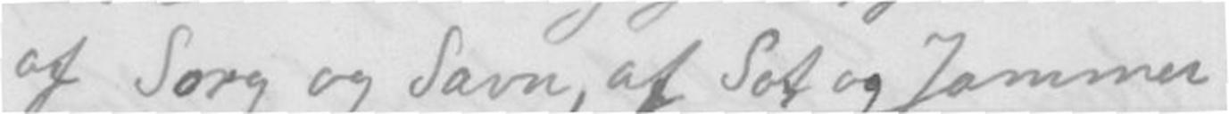af Sorg og Savn, af Sot og Jammer
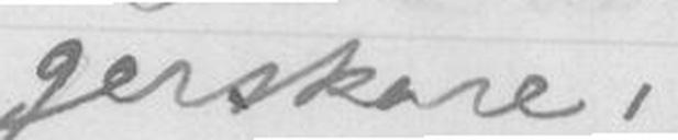gerskare,
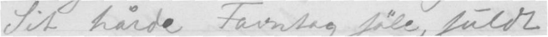Sit hårde Favntag føle fuldt
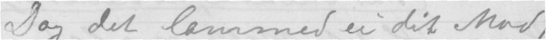Dog det lammed ei dit Mod,
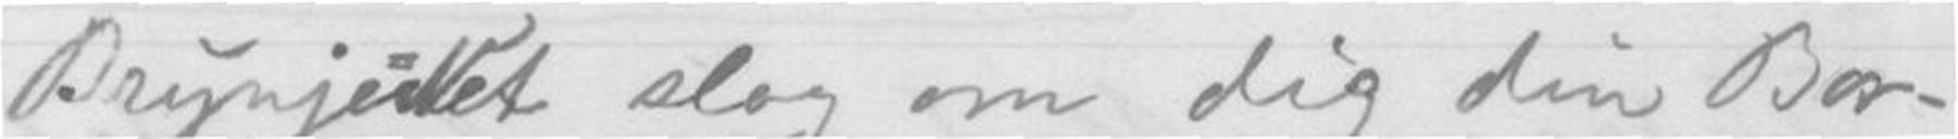Brynje=Net slog om dig din Bor-
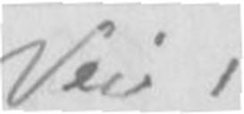Vei,
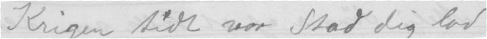Krigen tidt vor Stad dig lod
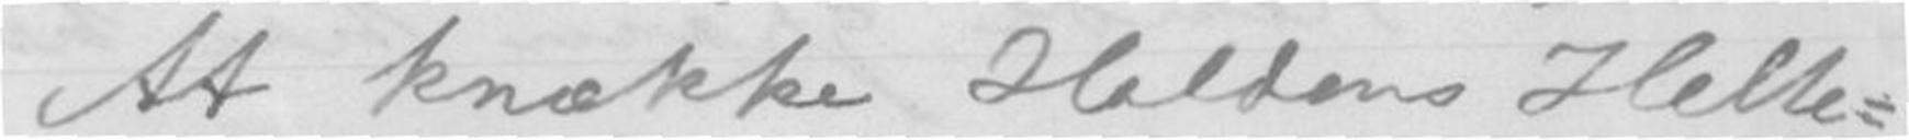At knække Haldens Helte-
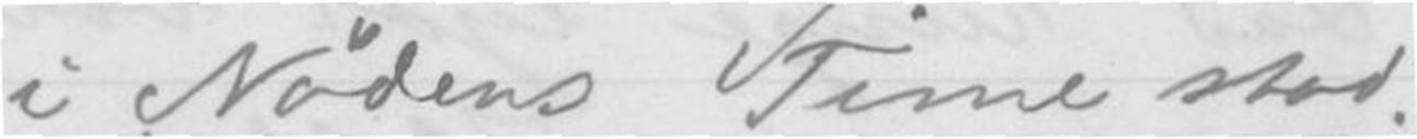i nødens Time stod.
